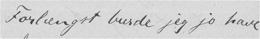Forlængst burde jeg jo have
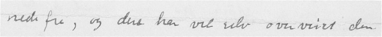nede fra, og dere har vel selv overveiet den
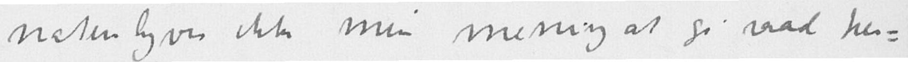naturligvis ikke min mening at gi raad her-
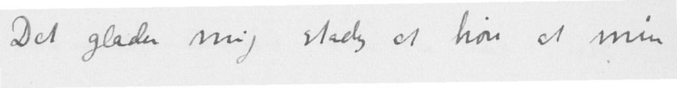Det glæder mig stadig at høre at min
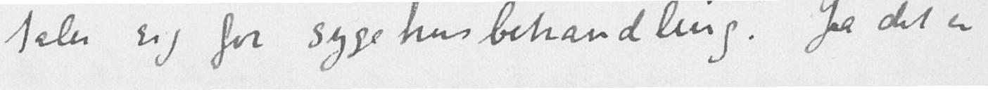taler sig for sygehusbehandling. Ja det er
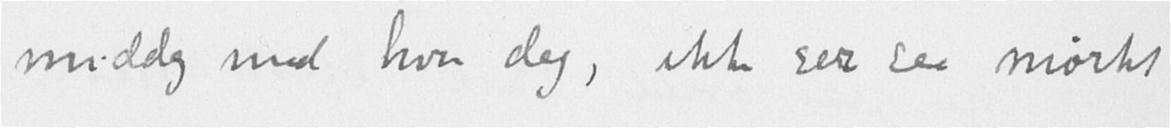middag med hver dag, ikke ser saa mørkt
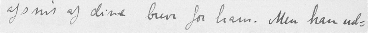afsnit af dine breve for ham. Men han ud-
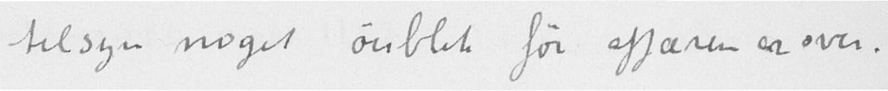tilsyn noget øieblik før affæren er over.
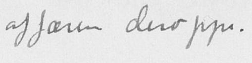affæren deroppe.
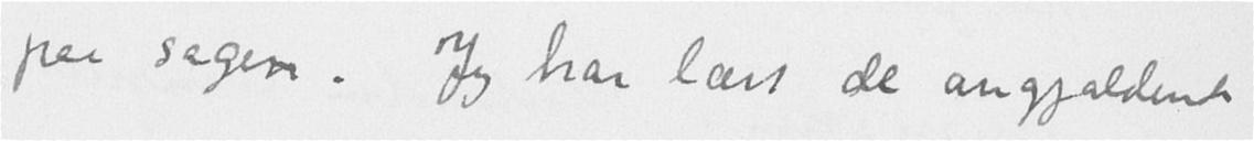paa sagen. Jeg har læst de angjældende
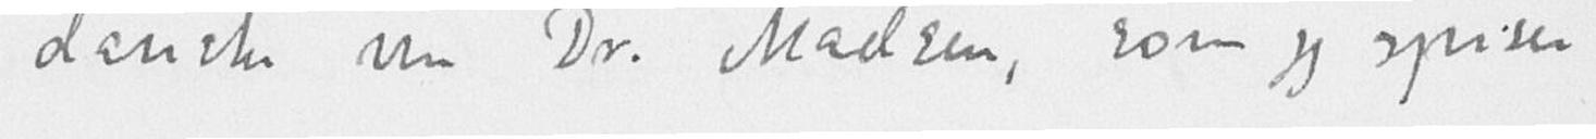danske ven Dr. Madsen, som jeg spiser
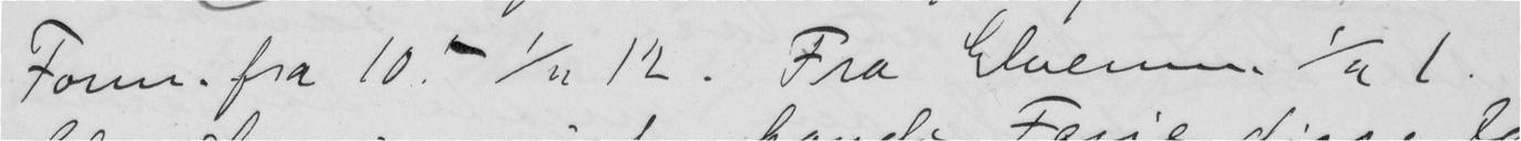Form. fra 10 - 1/2 12. Fra Elverum 1/2 1.
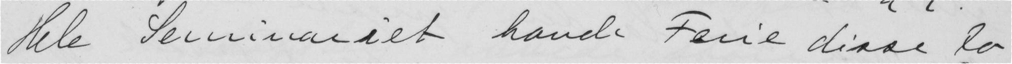Hele Seminariet havde Ferie disse to
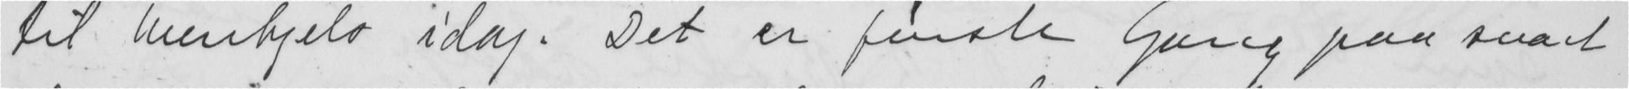til Wentzels idag. Det er første Gang paa snart
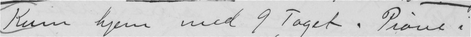Kom hjem med 9 Toget. Prøve i
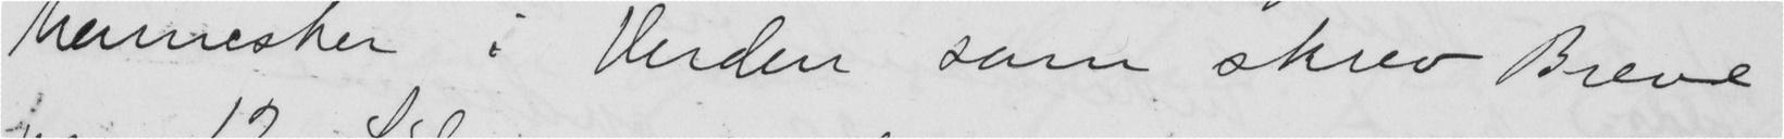Mennesker i Verden som skrev Breve
